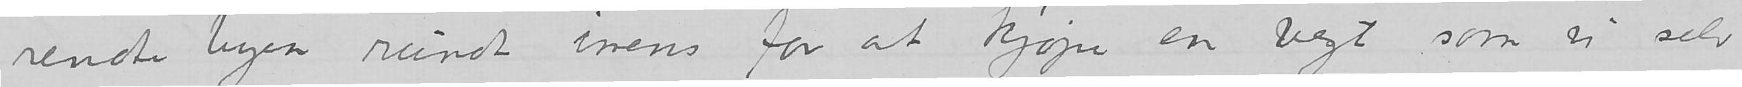rendte byen rundt imens for at kjøpe en vegt som vi selv
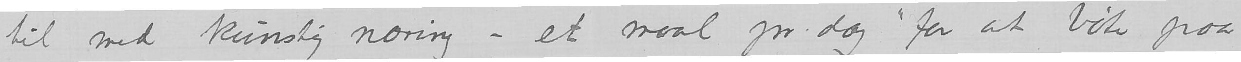til med kunstig næring – et maal pr. dag «for at bøte paa»
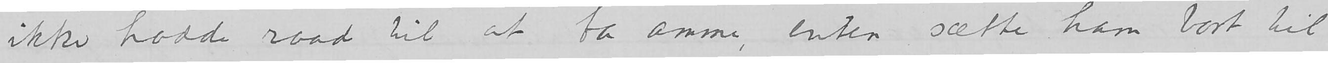ikke hadde raad til at ta amme, enten sætte ham bort til
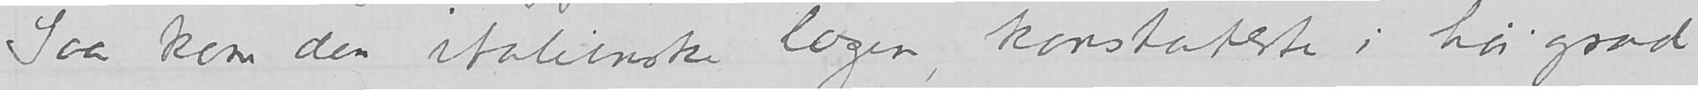Saa kom den italienske lægen, konstaterte i høi grad
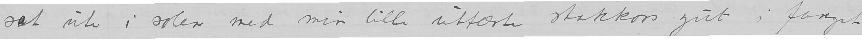sat ute i solen med min lille uttærte stakkars gut i fanget
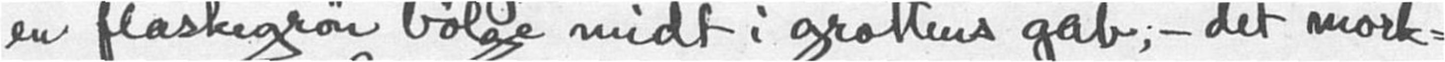en flaskegrøn bølge midt i grottens gab; - det mørk-
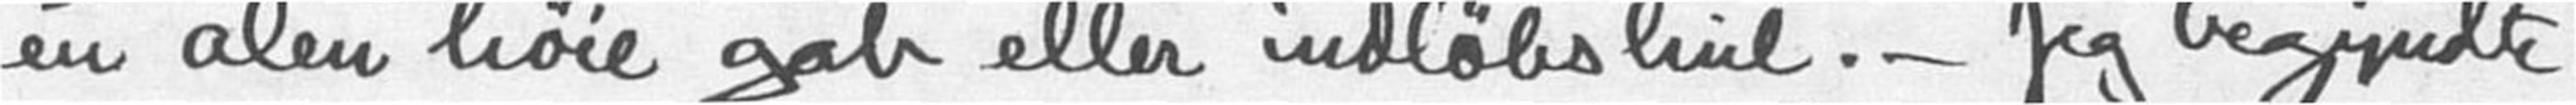en alen høie gab eller indløbshul. - Jeg begyndte
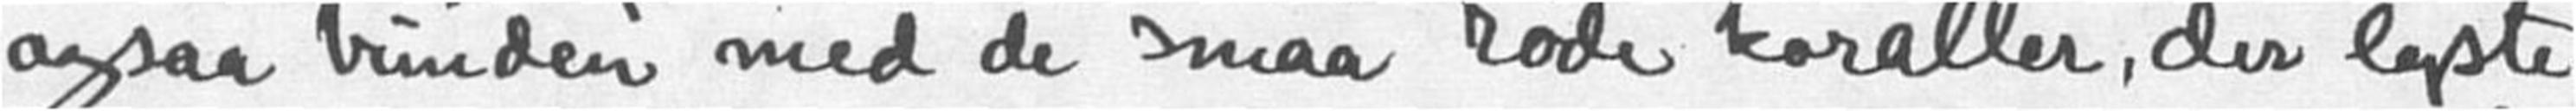ogsaa bunden med de smaa røde koraller, der lyste
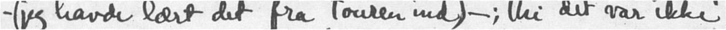- (jeg havde lært det fra touren ind) -: thi det var ikke
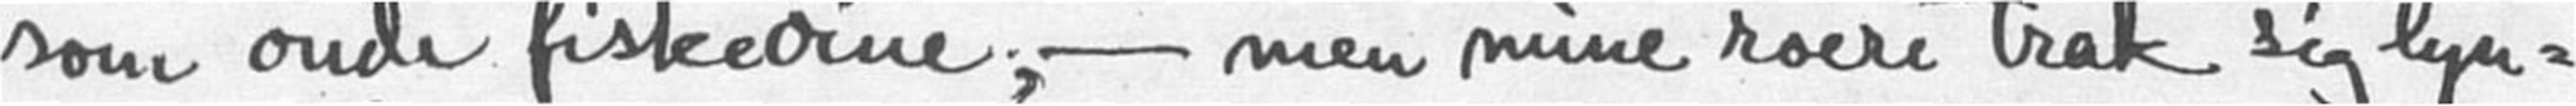som onde fiskeøine,- men mine roere trak sig lyn
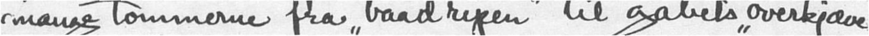mange tommerne fra "baadripen" til gabets "overkjæve"
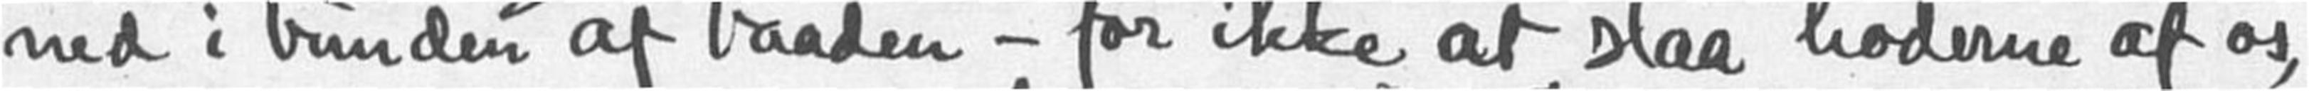ned i bunden af baaden - for ikke at slaa hoderne af
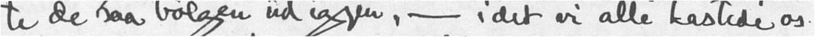te de saa bølgen ud igjen, - idet vi alle kastede os
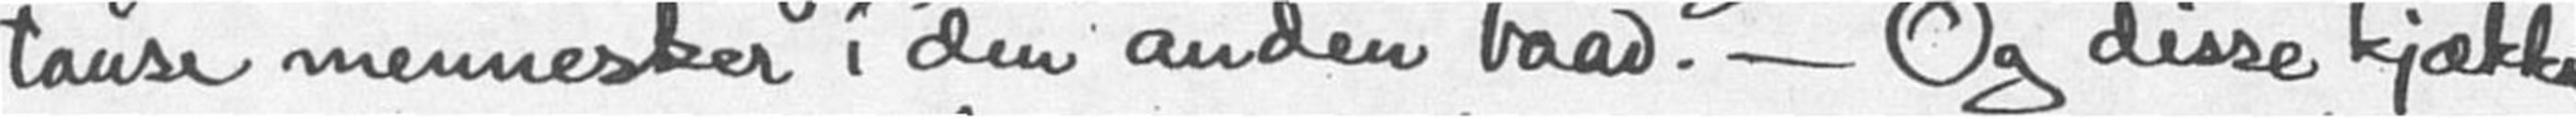tause mennesker i den anden baad, - Og disse kjække
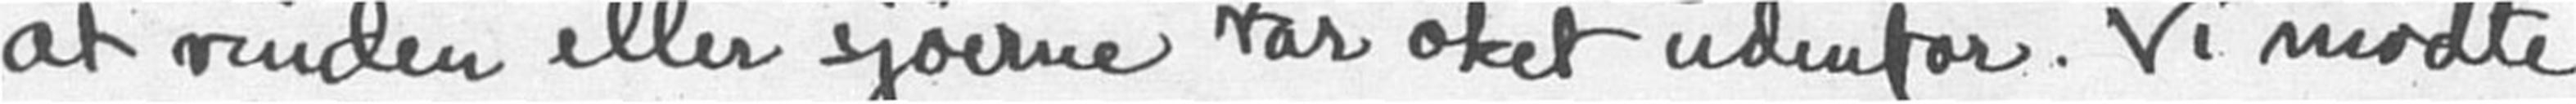at vinden eller sjøerne var øket udenfor. Vi mødte
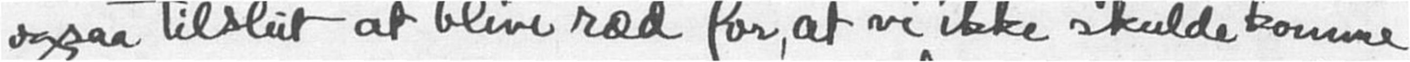ogsaa tilslut at blive ræd for, at vi ikke skulde komme
